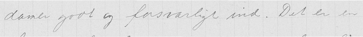damer godt og forsvarligt ind. Det er en
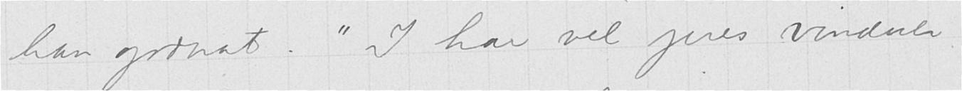han godnat. "I har vel jeres vinduer
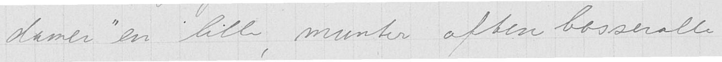damer "en lille, munter aftenbosseralle"
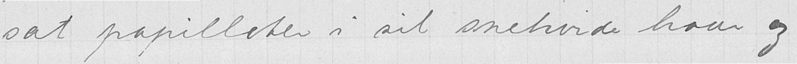sat papilloter i sit snehvide haar og
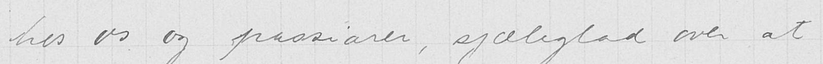hos os og passiarer, sjæleglad over at
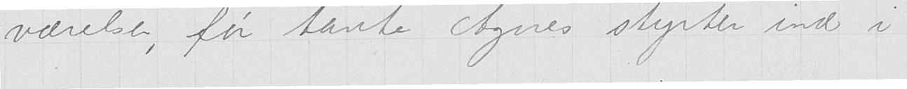værelser, før tante Agnes styrter ind i
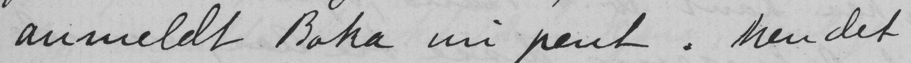anmeldt Boka mi pent. Men det
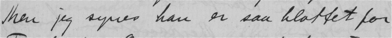Men jeg synes han er saa blottet for
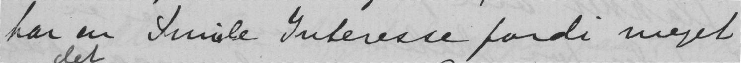har en Smule interesse fordi meget
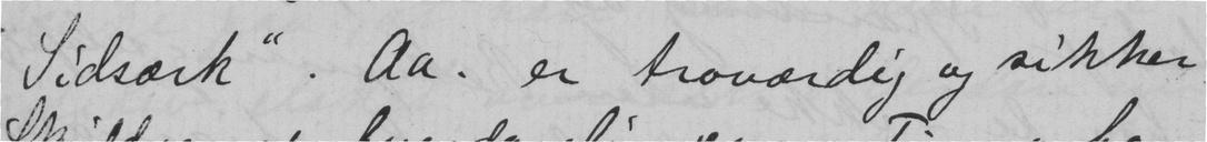Sidsærk". Aa. er troværdig og sikker
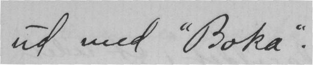ud med "Boka".
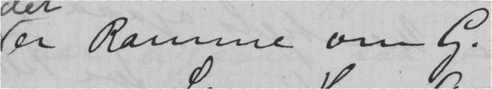er Ramme om G.
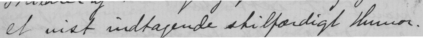et vist indtagende stilfærdigt Humor.
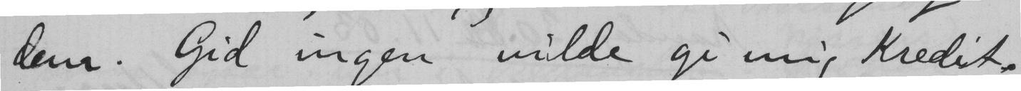dem. Gid ingen vilde gi mig Kredit.
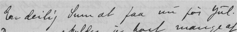En deilig Sum at faa nu før jul.
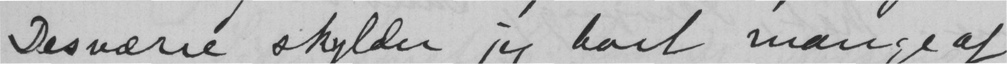Desværre skylder jeg bort mange af
